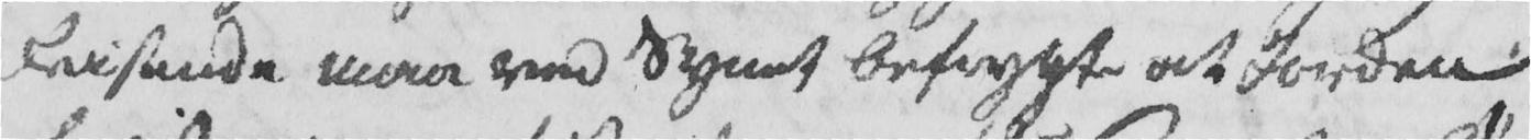Reisende maa ved Synet befrygte at Jorden
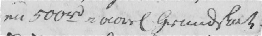nu 500 rd i aarl. Grundskat.
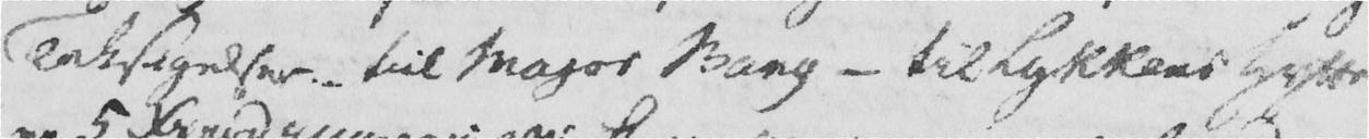Taksigelser. - til Major Bang - til Lykkens Hytte
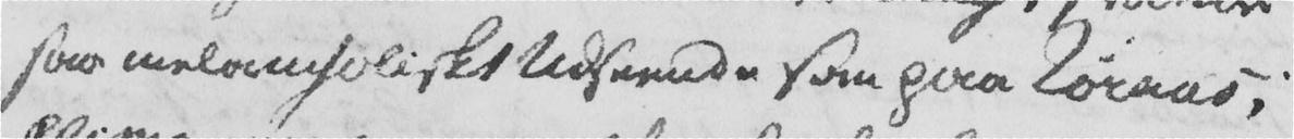saa melancholiskt Udseende som paa Røraas;
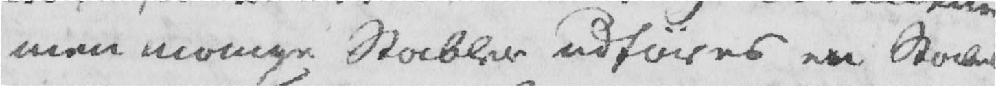men mange Stabler udføres en Stabel
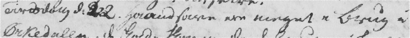Tirsdag d. 22. Haandsave ere meget i Brug i
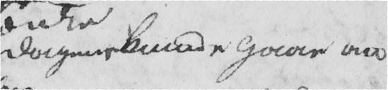Dagene kunde gaae an
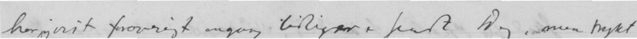her gjort forøvrigt engang tilsiger sendt. Dog men trykt
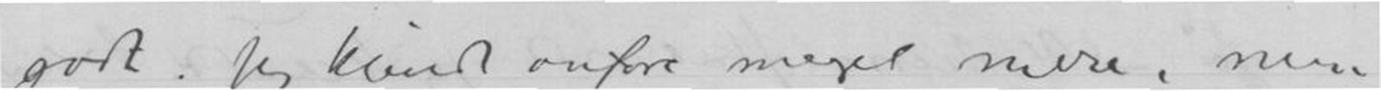godt. Jeg kunde anføre meget mere, men
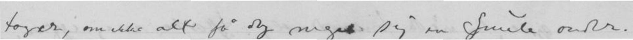tager, om ikke alt sa dog meget sig en Smule ander-
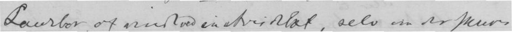Laurbær, af vindt ved en Avisdelat selv om der skrives
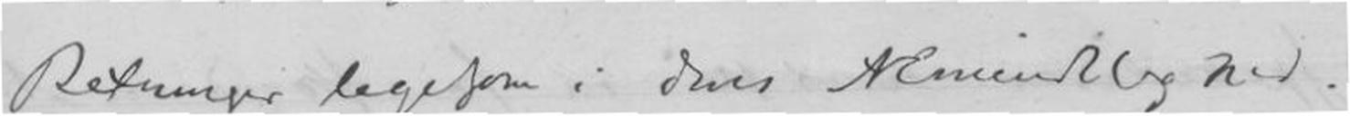Retninger ligesom i deres Almindelighed.
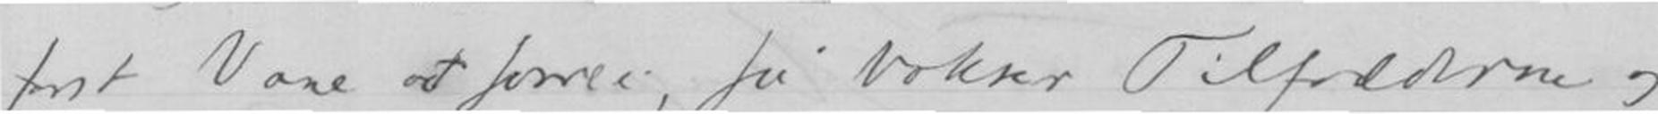det først Vore at svare i, så vokser Tilfælderne og
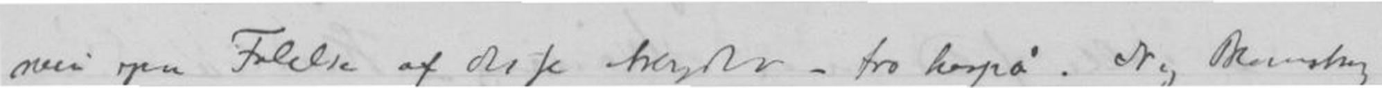min egen Følelse af disse Arbejder - tro herpå. Ny Blomstring
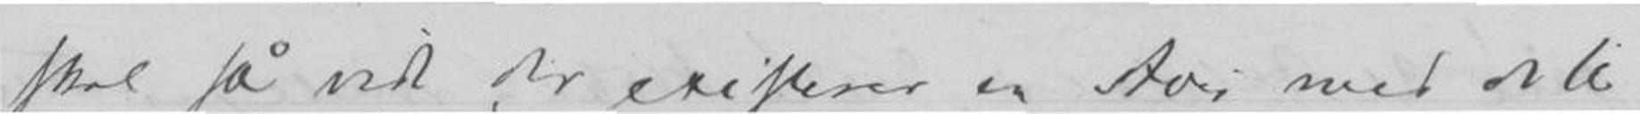skal så vidt der existerer en Avis med dette
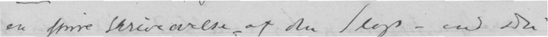en større skriveøvelse af den Slags - end du
